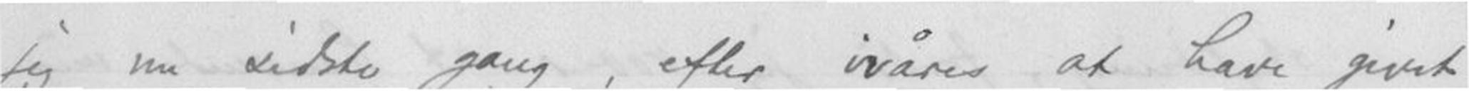jeg nu sidste gang, efter ivåres at have givet
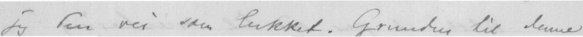jeg den vei som lukket. Grunden til denne
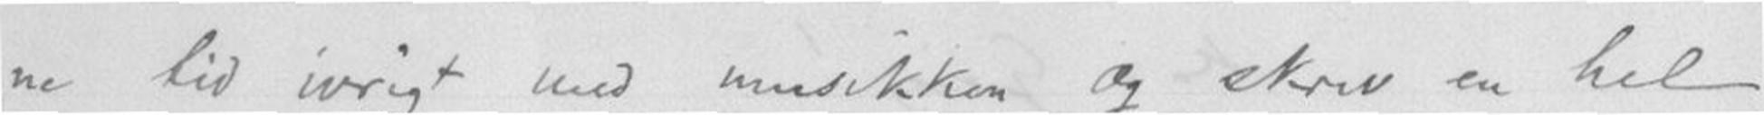ne tid ivrigt med musikken og skrev en hel
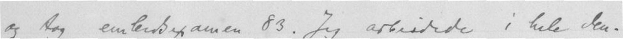og tog embedsexamen 83. Jeg arbeidede i hele den-
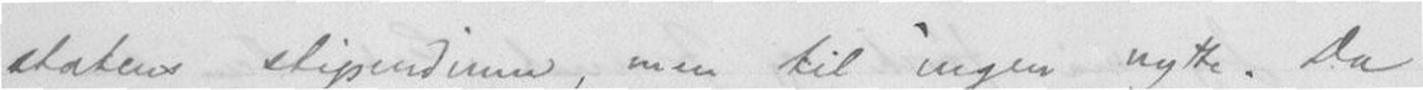statens stipendium, men til ingen nytte. Da
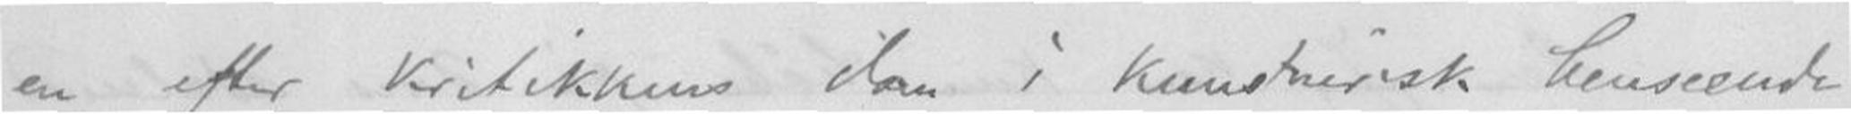en efter kritikkens dom i kunstnerisk henseende
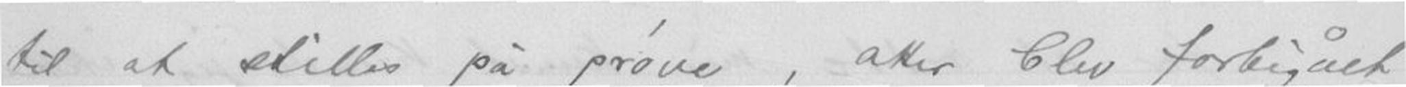til at stilles på prøve, atter blev forbigået
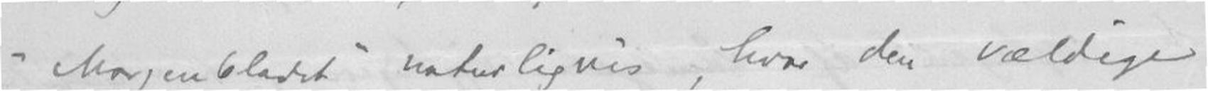"Morgenbladet" naturligvis, hvor den vældige
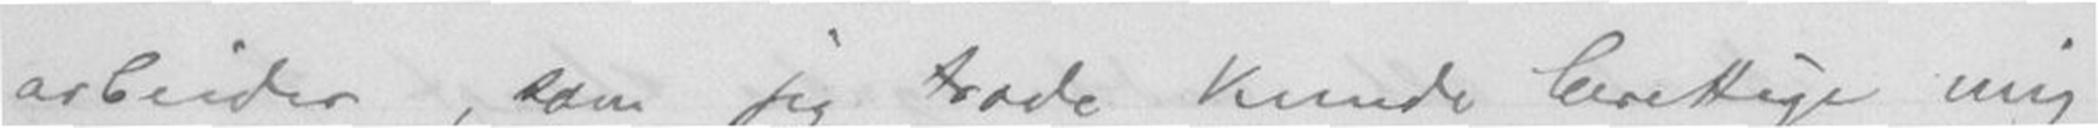arbeider, som jeg torde kunde berettige mig
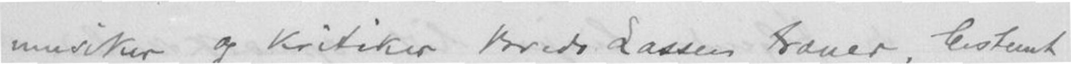musiker og kritiker Brede Lassen Bauer, bestemt
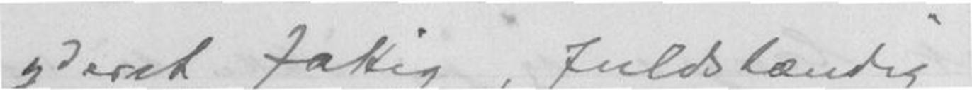yderst fattig, fuldstændig
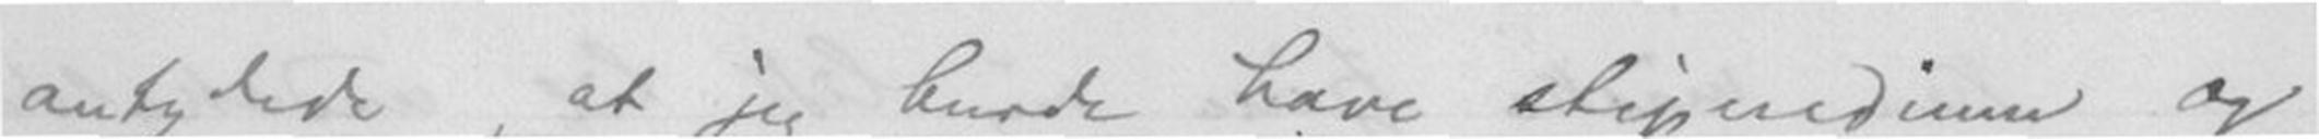antydede, at jeg burde have stipendium og
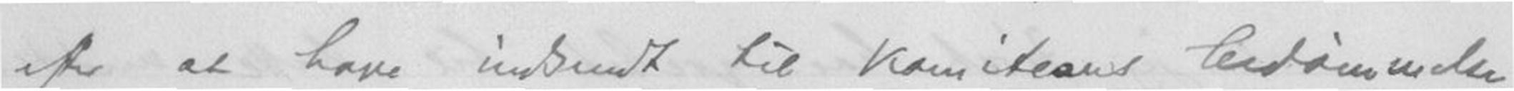efter at have indsendt til komiteens bedømmelse
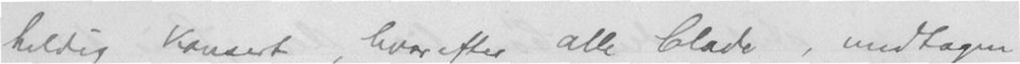heldig konsert, hvorefter alle blade, undtagen
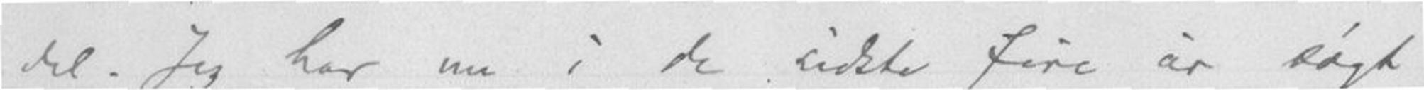del. Jeg har nu i de sidste fire år søgt
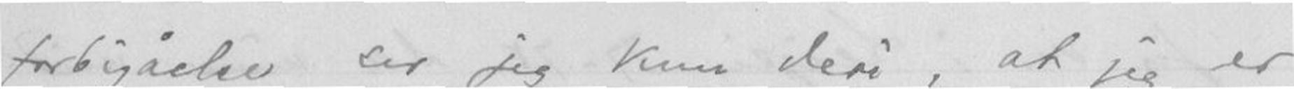forbigåelse ser jeg kun deri, at jeg er
